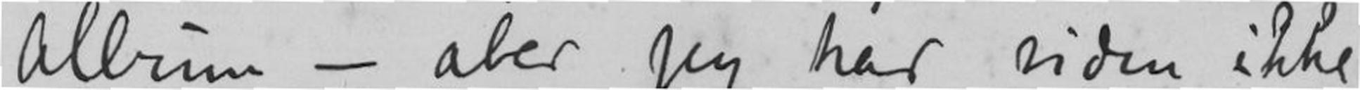Album - aber jeg har siden ikke
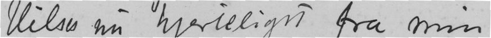Hilses nu hjertelig fra min
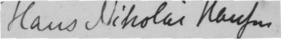Hans Nikolai Hansen
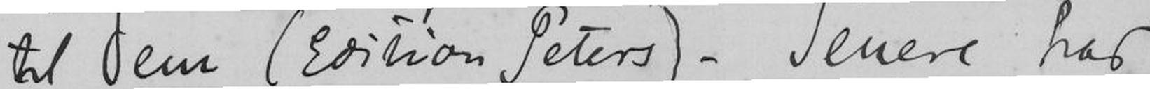til Dem (Edition Peters) - Senere har
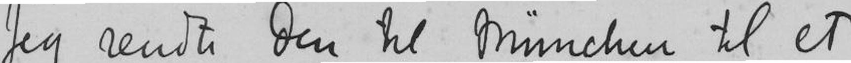Jeg sendte den til München til et
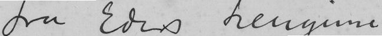fra Eders hengivne
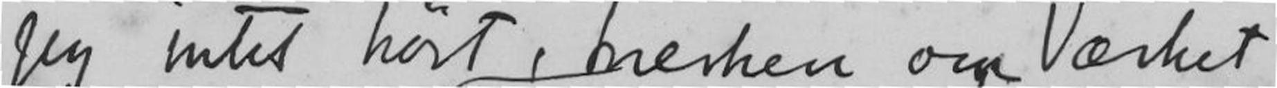jeg intet hørt , hverken om Værket
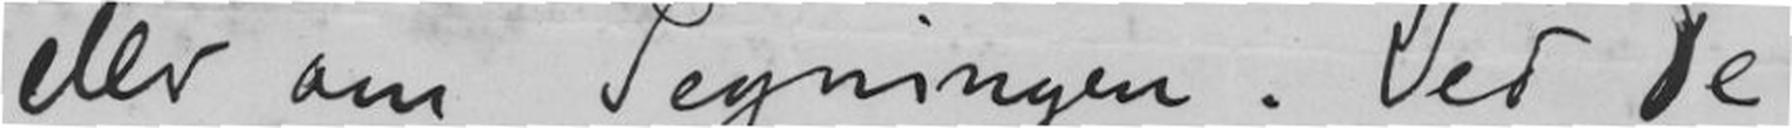eller om Tegningen. Ved de
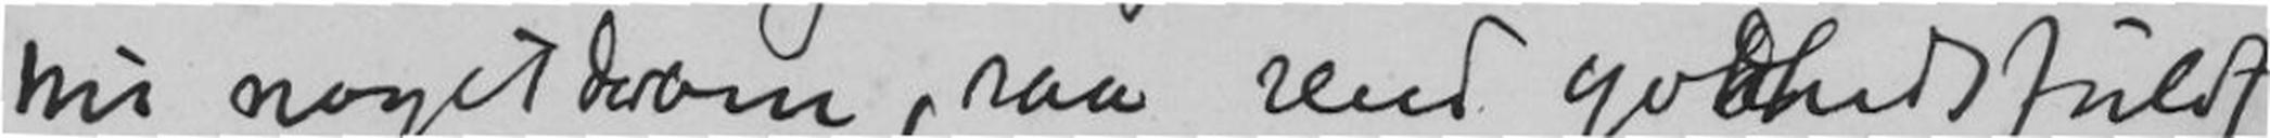nu noget derom, saa send godhedsfult
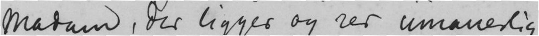Madam, der ligger og ser umanelig
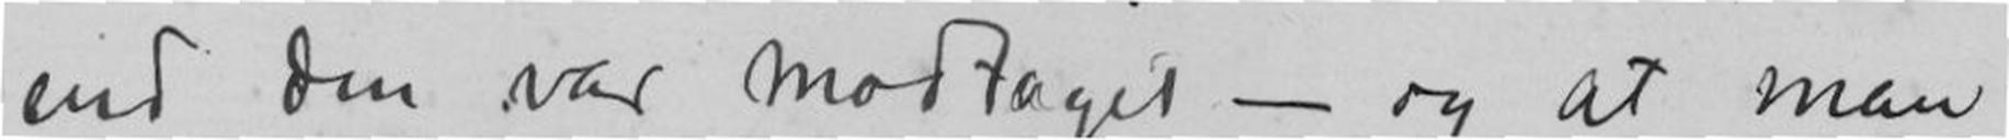end den var modtaget - og at man
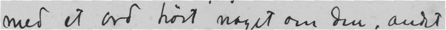med et ord hørt noget om den, andet
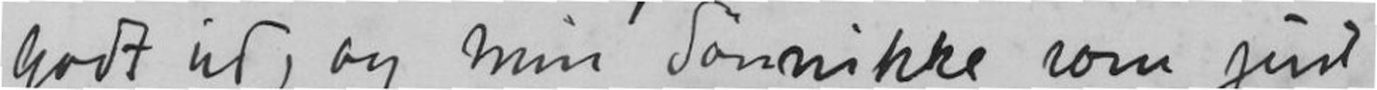godt ud, og min Sønnikke som just
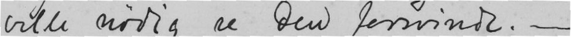ville nødig se Den forsvinde.
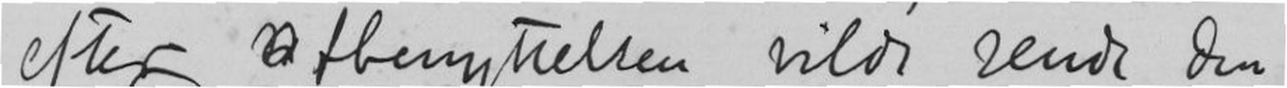efter afbemytelsen vilde sende den
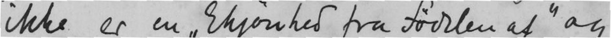ikke er en Skjønhed fra fødslen af "og
